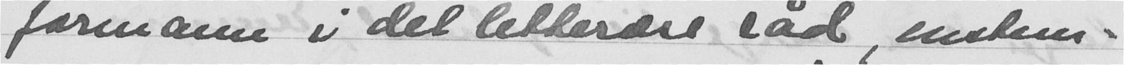formann i det litterære råd, enstem-
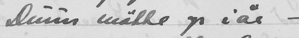Duun måtte op iår -
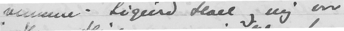"vennene" Sigurd Hoel og mig var
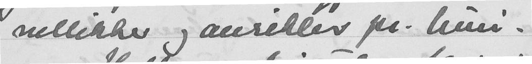nellikker og anriller pr. juni.
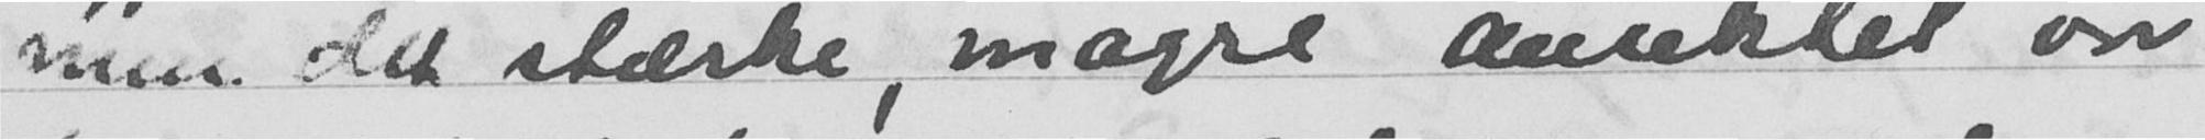men det stærke, magre ansiktet var
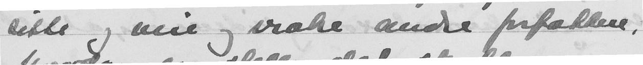sitte og veie og vrake andre forfattere,
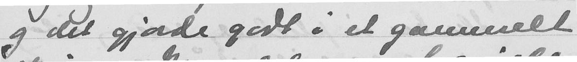og det gjorde godt i et gammelt
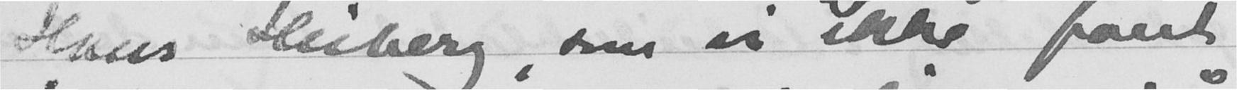Hans Heiberg, som vi ikke fant
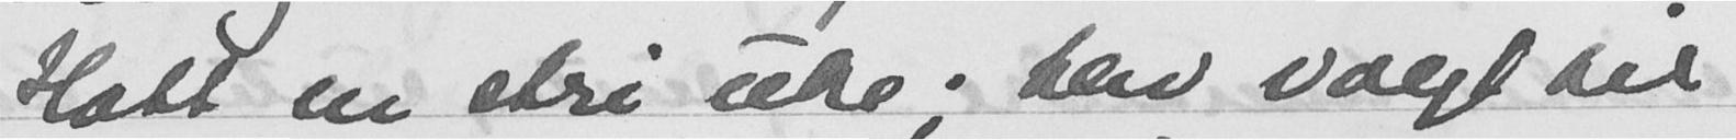Hatt en stri uke; blev valgt til
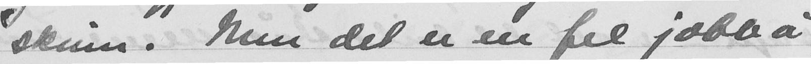skinn. Men det er en fel jobb å
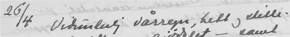26/4 Vidunderlig vårregn, hett og stille.
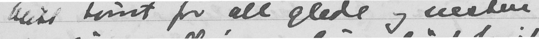blitt tømt for all glede og nesten
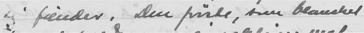sig fiender. Den første, som blomstret
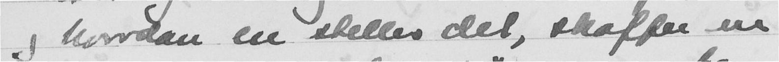og hvordan en stiller det, skaffer en
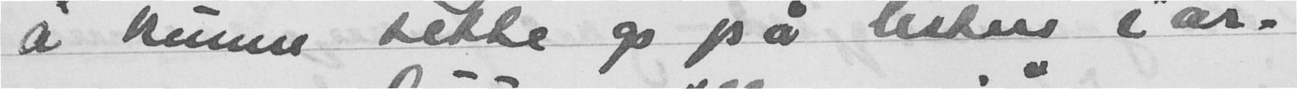å kunne sette op på listen i år.
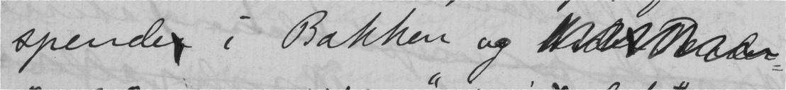spende i Bakken og
